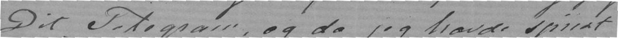Dit Telegram, og da jeg havde spiist
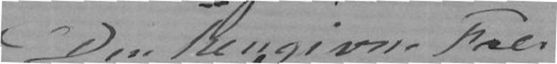Din hengivne Faer
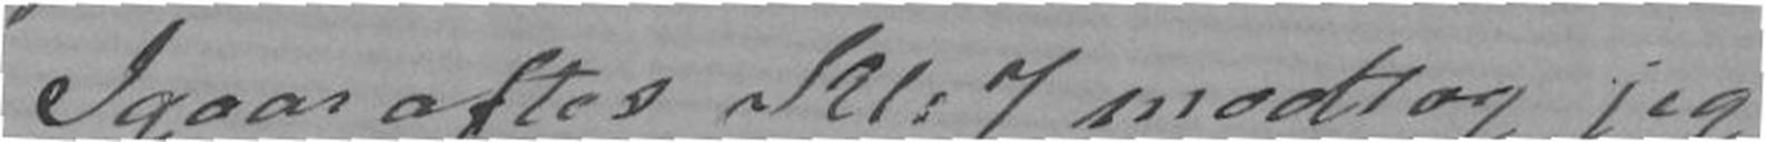Igaaraftes Kl:7 modtog jeg
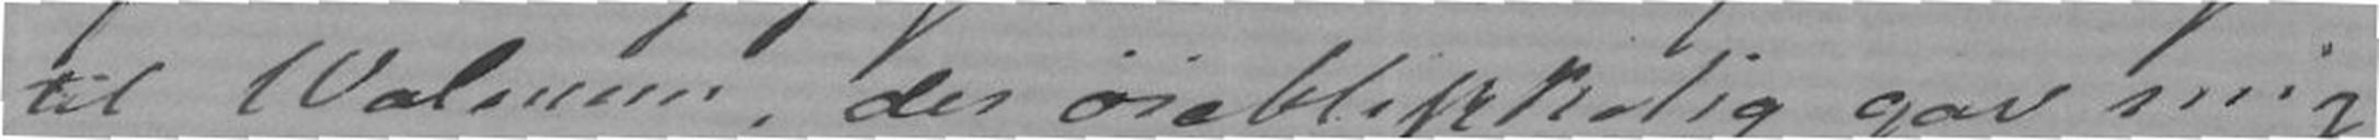til Walman, der øieblikkelig gav mig
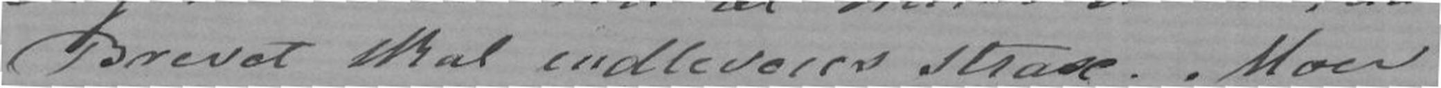Brevet skal indleveres strax. Moer
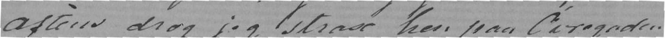Aftens drog jeg strax hen paa Øvregaden
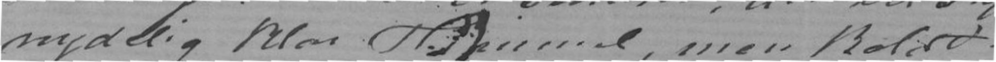nydelig klar Himmel, men koldt.
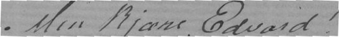Min kjære Edvard!
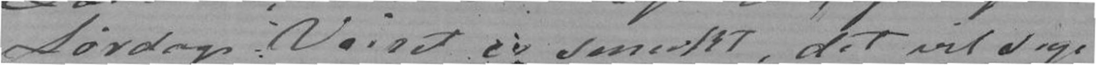Lørdag. Veiret er smukt, det vil sige
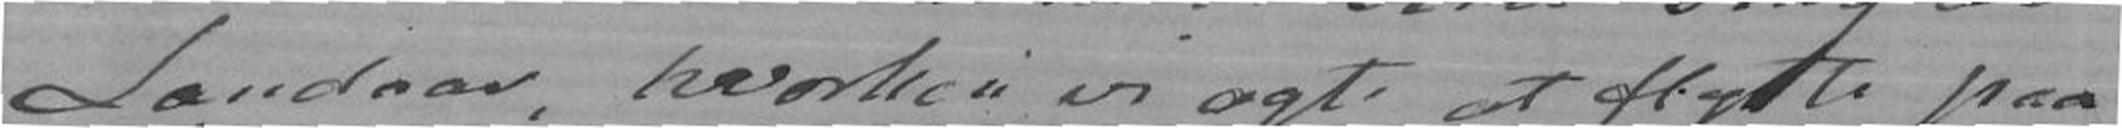Landaas, hvorhen vi agte at flytte paa
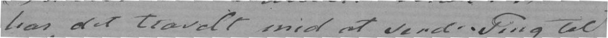har det travelt med at sende Ting til
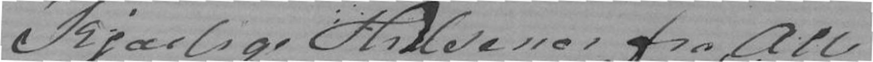Kjærligen Hilsener fra Alle
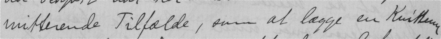mitterende Tilfælde, som at lægge en Kvittering
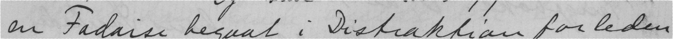en Fadaise begaat i Distraktion forleden
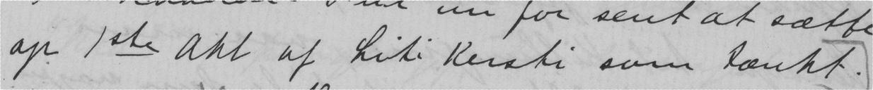op 1ste Akt af Liti Kersti som tænkt.
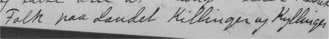Folk paa Landet Killinger og Kyllinger
